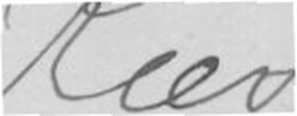Ræd
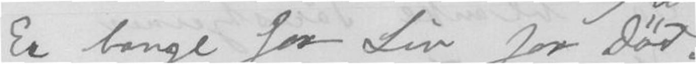Er bange for Liv for Død. -
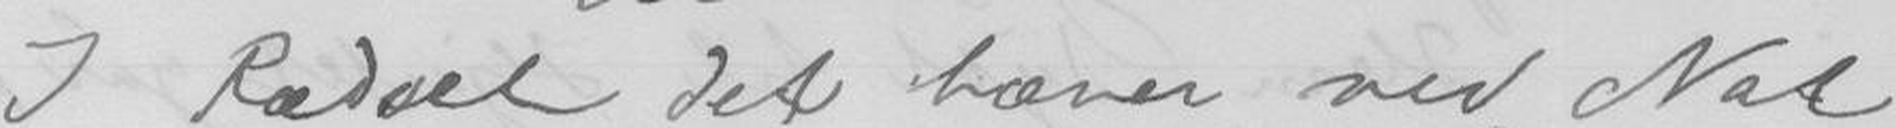I Rædsel det bæver ved Nat
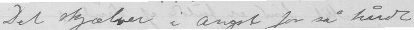Det skjælver i angst for så hårt
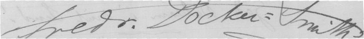Fredr. Döcker=Smith
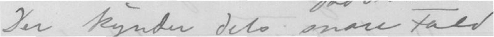Der kynder dets snare Fald.
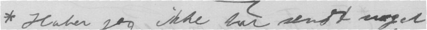* Håber jeg ikke har sendt noget
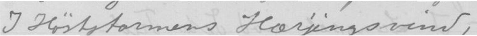I Høststormens Hærjingsvind,
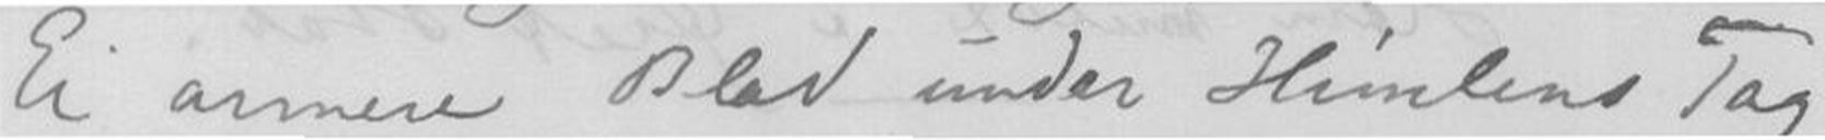Ei annen Blad under Himlens Tag
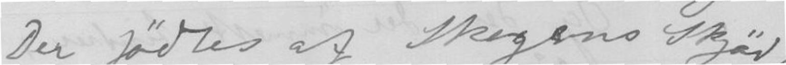Der fødtes af Skogens Skjød!
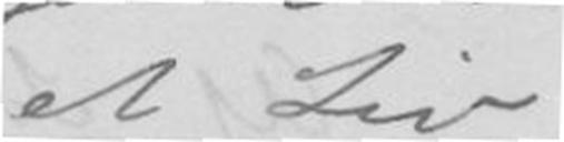et Liv
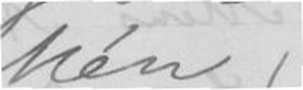Mén,
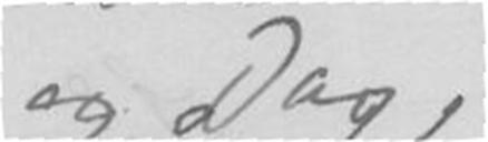og Dag, -
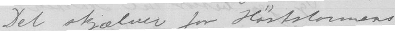Det skjælver for Høststormers
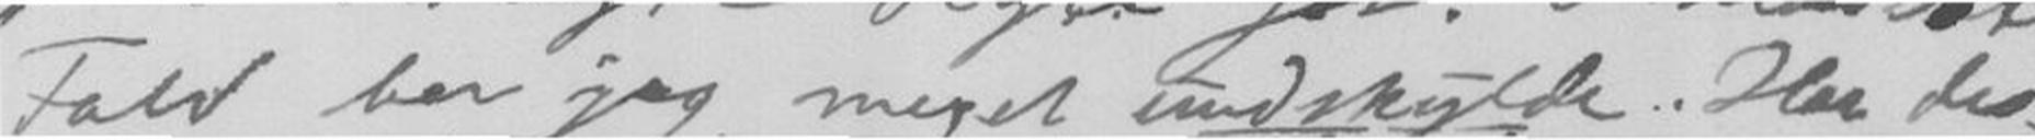Fald ber jeg meget undskylde. Har des
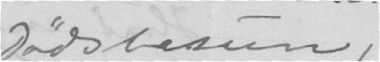Dødsbasun,
